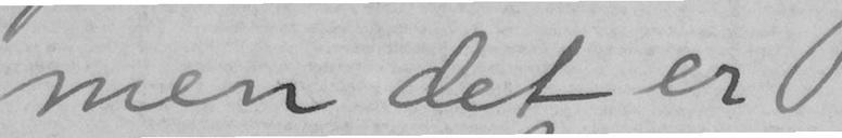men det er
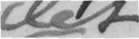det
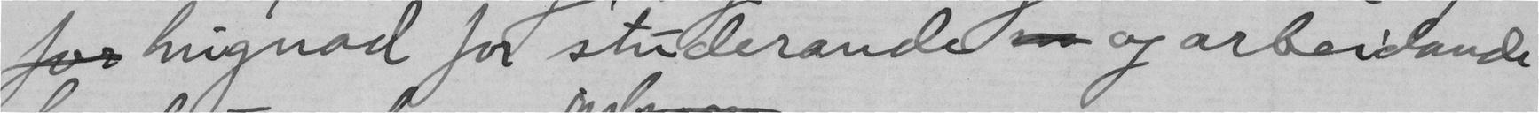for hugnad for studerande - og arbeidande
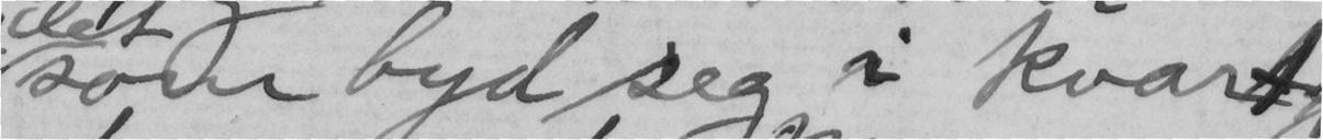som byd seg i kvart
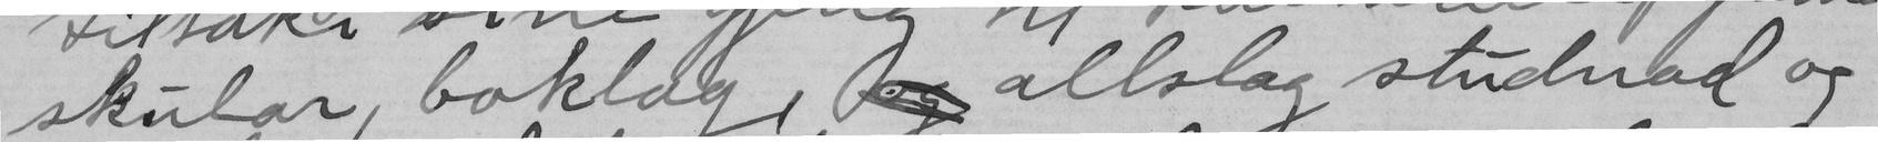skular, boklag, og allslag studnad og
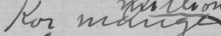Kor mange
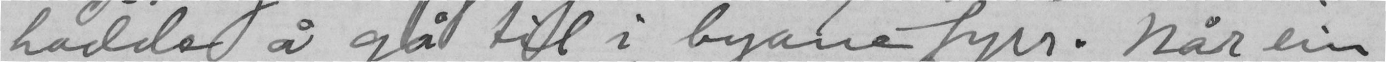hadde å gå til i byane fyrr. Når ein
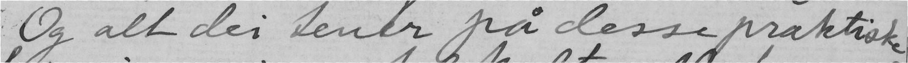Og alt dei tener på desse praktiske
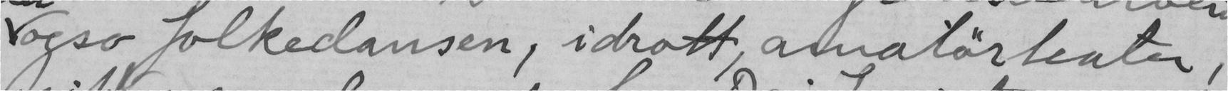ogso folkedansen, idrott, amatørteater,
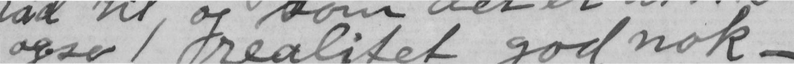ogso 1 realitet god nok -
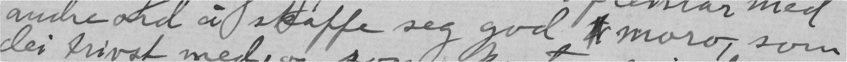andre ord å skaffe seg god moro, som
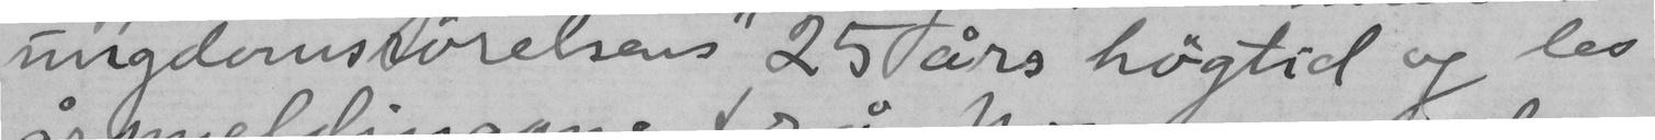ungdomsrørelsens" 25 års høgtid og les
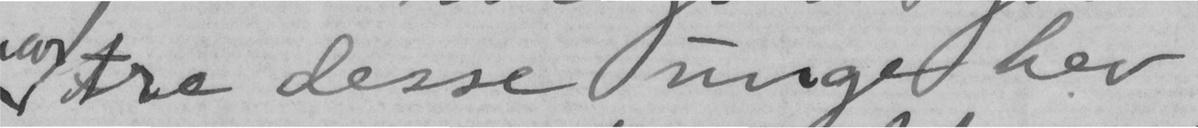tre desse unge hev
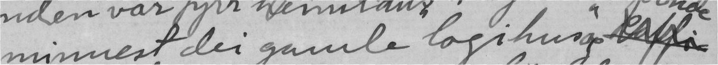minnest dei gamle logihus og kaffi-
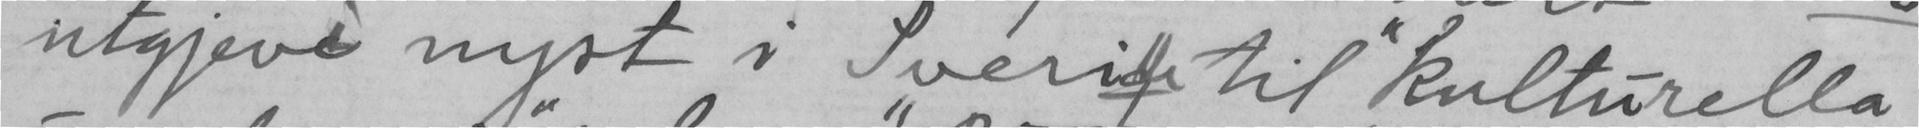utgjeve nyst i Sverige til "kulturella
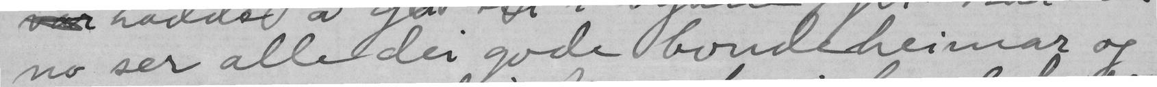no ser alle dei gode bondeheimar og
